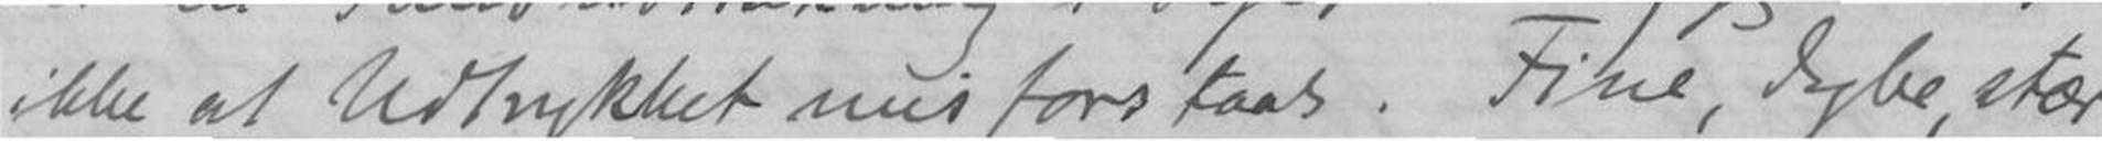ikke at Udtrykket misforstaas. Fine, dybe, stær-
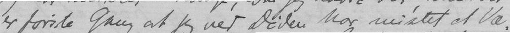er første Gang at jeg ved Døden har mistet et Væ-
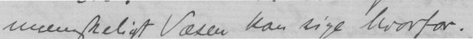engheligt Væsen kan sige hvorfor.
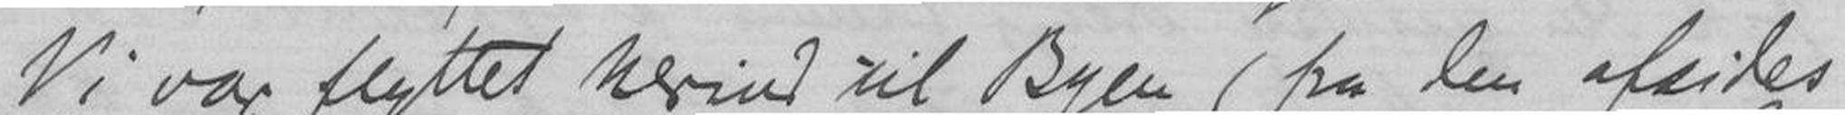Vi var flyttet herind til Byen (fra den afsides
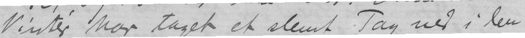Vinter har taget et slemt Tag ned i den
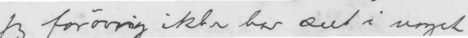jeg forøvrig ikke har seet i noget
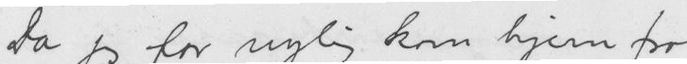Da jeg for nylig kom hjem fra
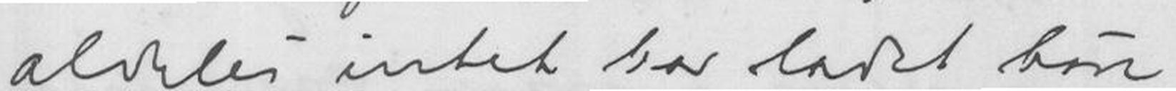aldeles intet har ladet høre
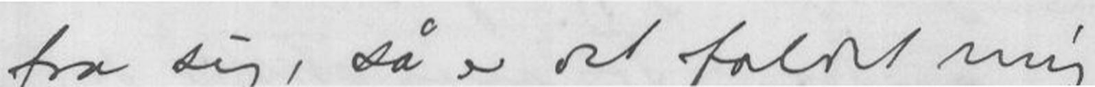fra sig, så er det faldet mig
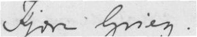Kjære Grieg!
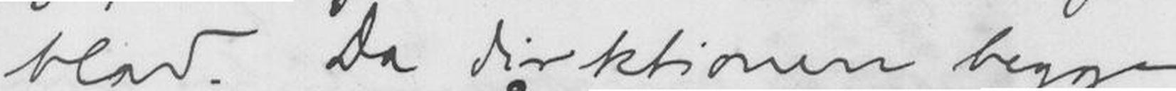blad. Da diriktionen begge
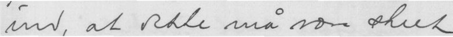ind, at dette må være skeet
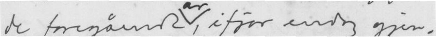de foregående år, ifjor endog gjen-
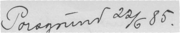Porsgrund 22/6 85.
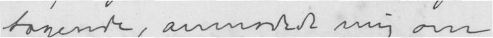tagende, anmodede mig om
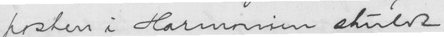posten i Harmonien skulde
